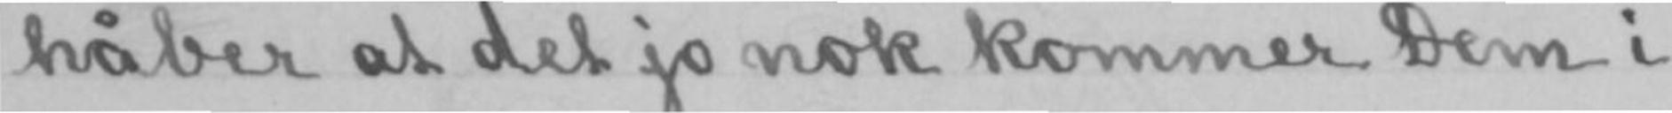håber at det jo nok kommer Dem i
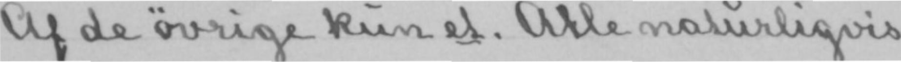Af de øvrige kun et. Alle naturligvis
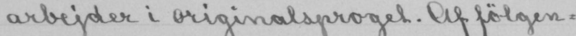arbejder i originalsproget. Af følgen-
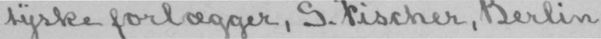tyske forlægger, S. Fischer, Berlin,
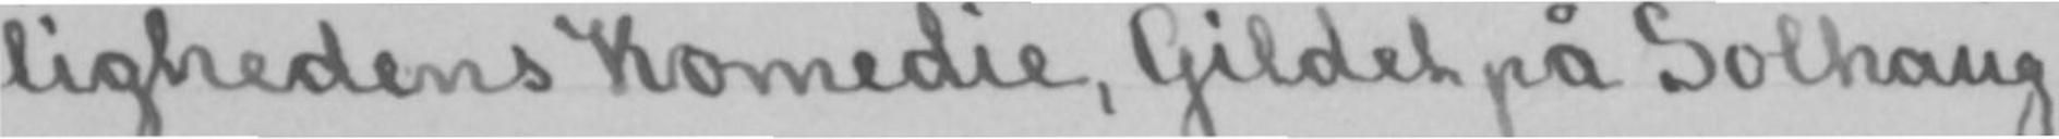lighedens Komedie, Gildet på Solhaug,
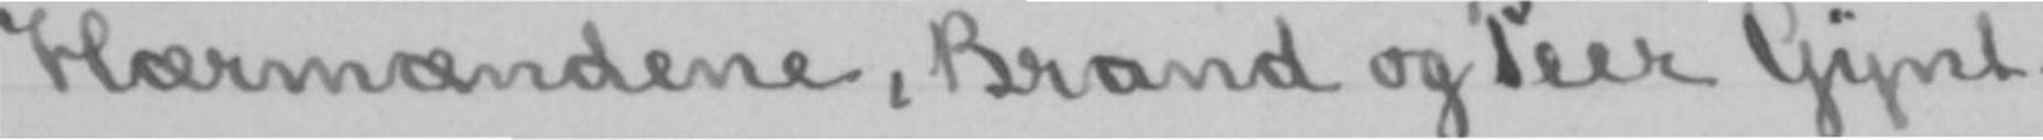Hærmændene, Brand og Peer Gynt.
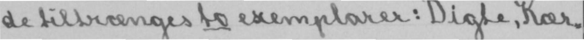de tiltrænges to exemplarer: Digte, Kær-
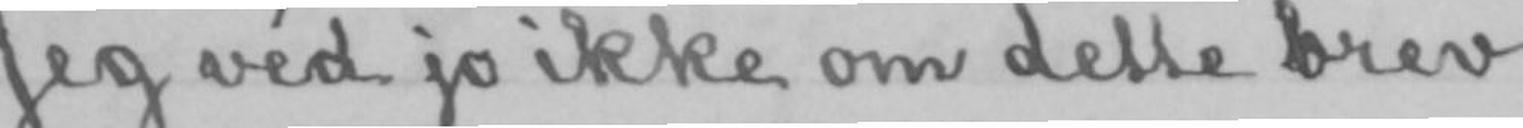Jeg véd jo ikke om dette brev
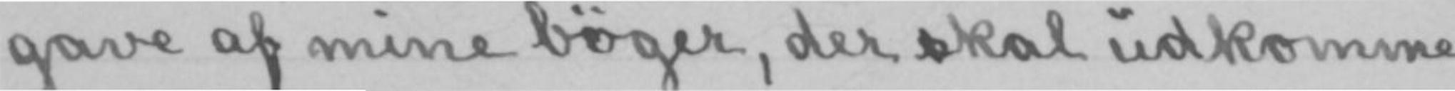gave af mine bøger, der skal udkomme
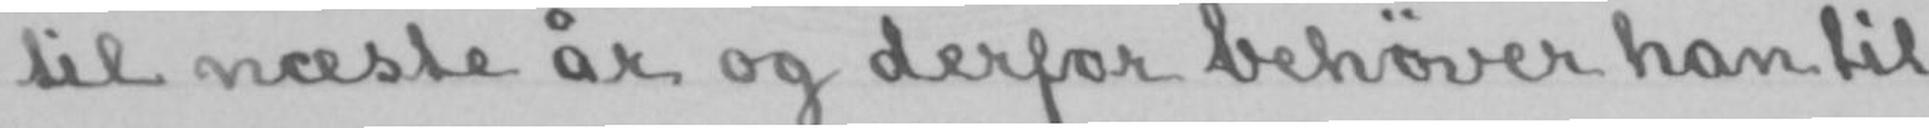til næste år og derfor behøver han til
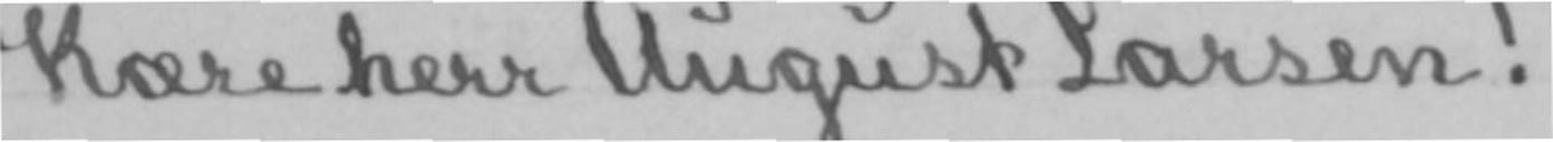Kære herr August Larsen!
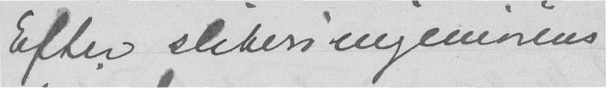Efter sliberiingeniørens
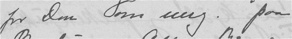for Don om mrg. paa
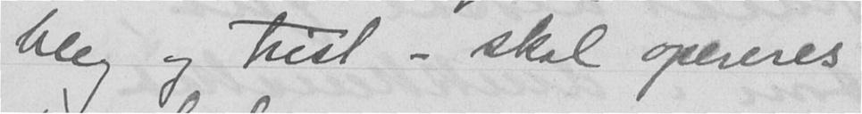bleg og trist - skal opereres
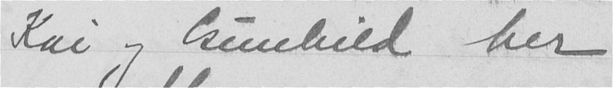Kai og Gunhild her
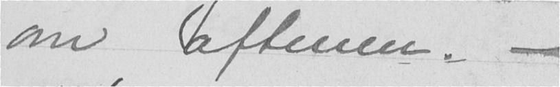om aftenen. -
